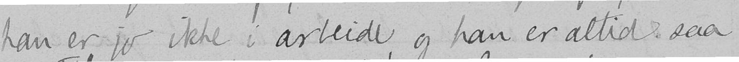han er jo ikke i arbeide, og han er altid saa
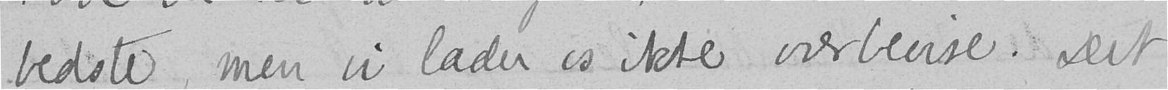bedste, men vi lader os ikke overbevise. Det
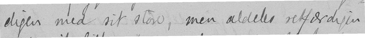digen med sit store, men aldeles retfærdigen
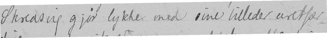Skredsvig gjør lykke med sine billeder uretfer-
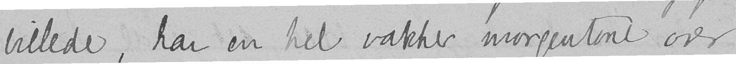billede, har en hel vakker morgentone over
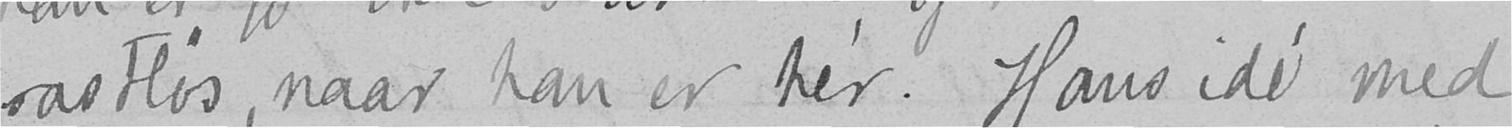rastløs naar han er her. Hans idé med
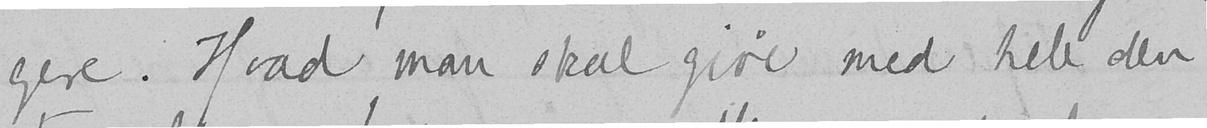gere. Hvad man skal gjøre med hele den
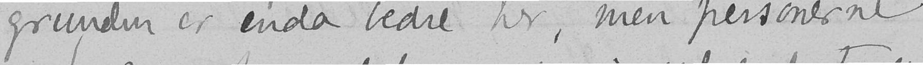grunden er enda bedre her, men personerne
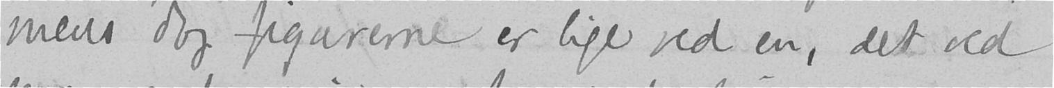mens dog figurerne er lige ved en, det ved
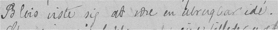Blois viste sig at være en ubrugbar idé.
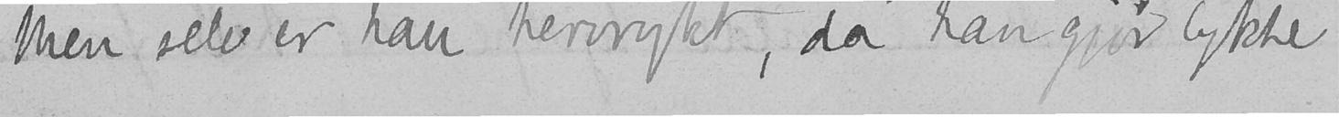Men selv er han henrykt, da han gjør lykke
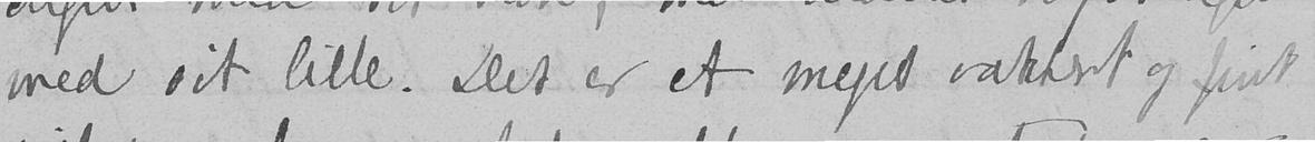med sit lille. Det er et meget vakkert og fint
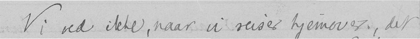Vi ved ikke, naar vi reiser hjemover, det
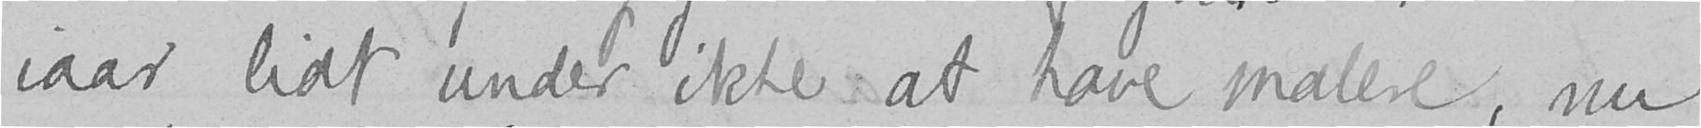iaar lidt under ikke at have malere, nu
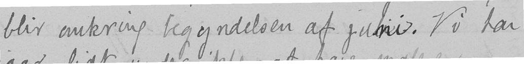blir omkring begyndelsen af juni. Vi har
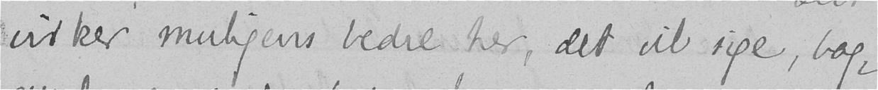virker muligens bedre her, det vil sige, bag-
